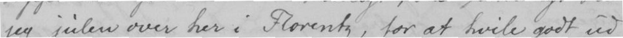jeg julen over her i Florentz, for at hvile godt ud
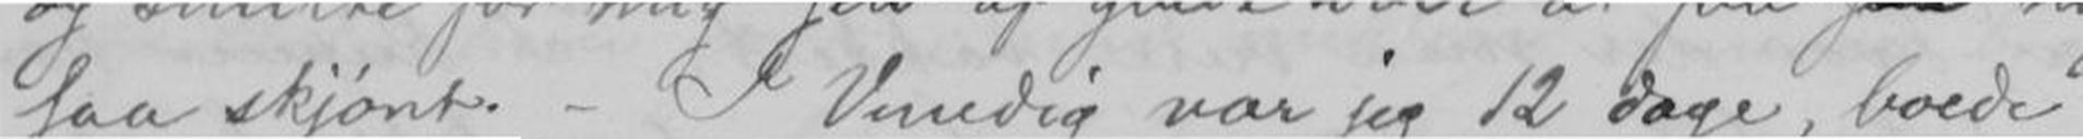saa skjønt. - I Venedig var jeg i 12 dage, boede
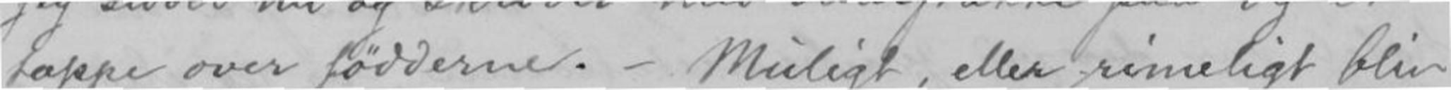tæppe over fødderne. - Muligt, eller rimeligt blir
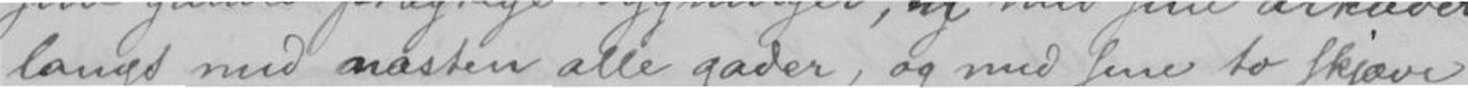langst med næsten alle gader, og med sine to skjæve
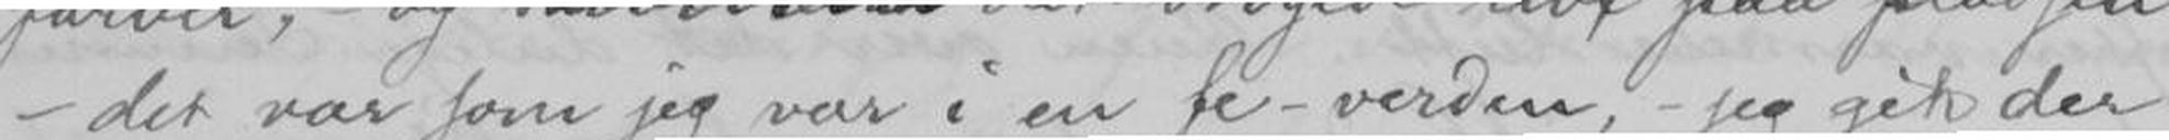- det var som jeg var i en fe-verden, - jeg gik der
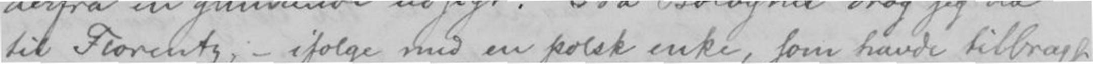til Florentz, - ifølge med en polsk enke, som havde tilbragt
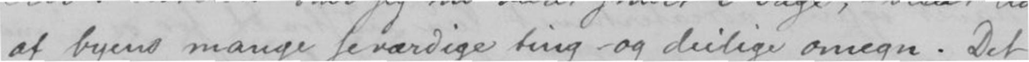af byens mange seværdige ting og deilige omegn. Det
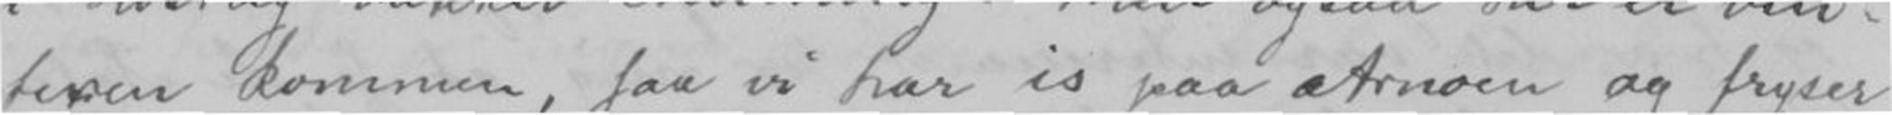teren kommen, saa vi har is paa Arnoen og fryser
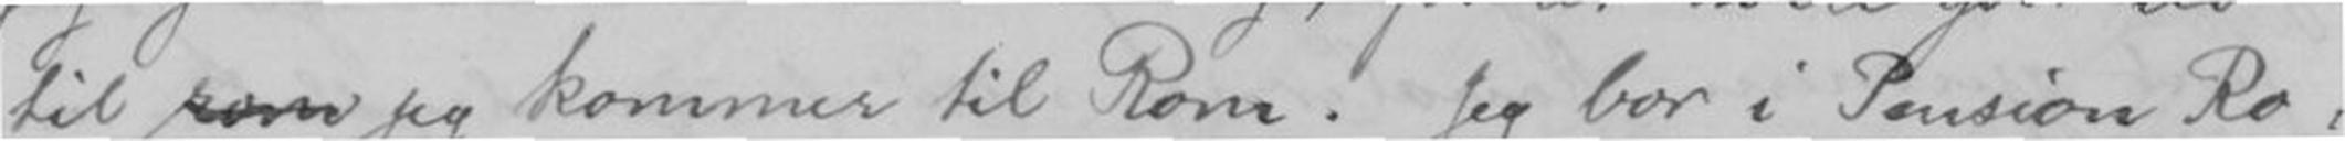til jeg kommer til Rom. Jeg bor i Pension Ro-
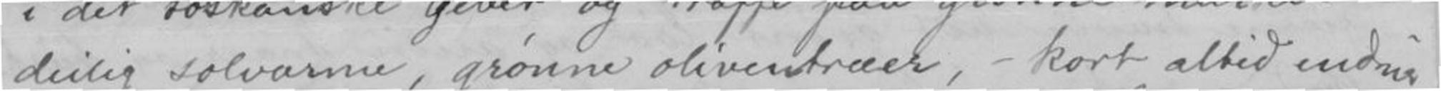deilig solvarme, grønne oliventræer, - kort altid endnu
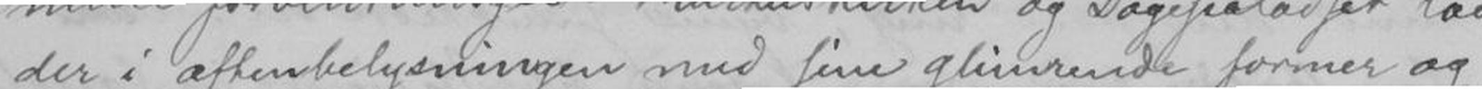der i aftenbelysningen med sine glimrende former og
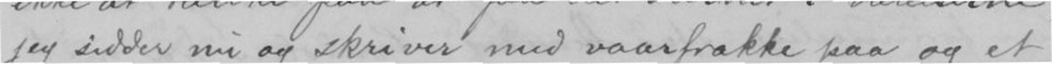jeg sidder nu og skriver med vaarfrakke paa og et
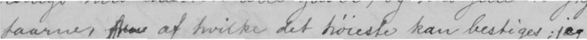taarne, af hvilket det høieste kan bestige; jeg
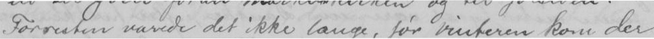Forresten varede det ikke længe, før vinteren kom der
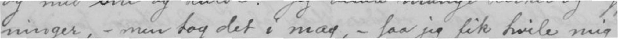ninger, - men tog det i mag,- saa jeg fik hvile mig
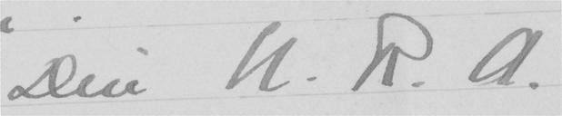Din N. R. A.
